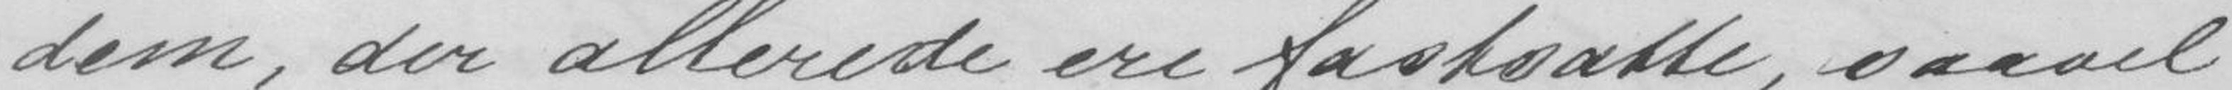dem, der allerede ere fastsatte, saavel
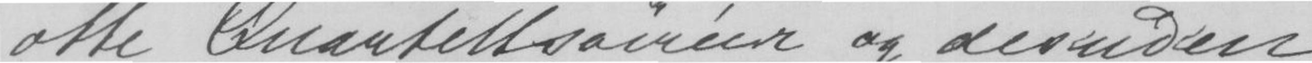otte Quartettsoiréer og desuden
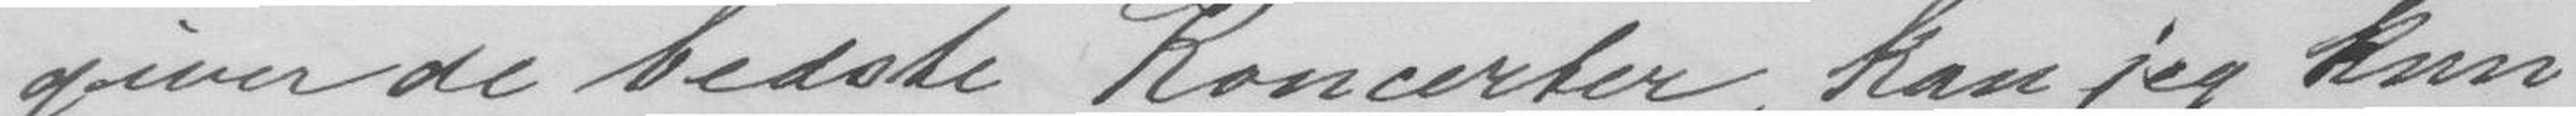giver de bedste Koncerter, kan jeg kun
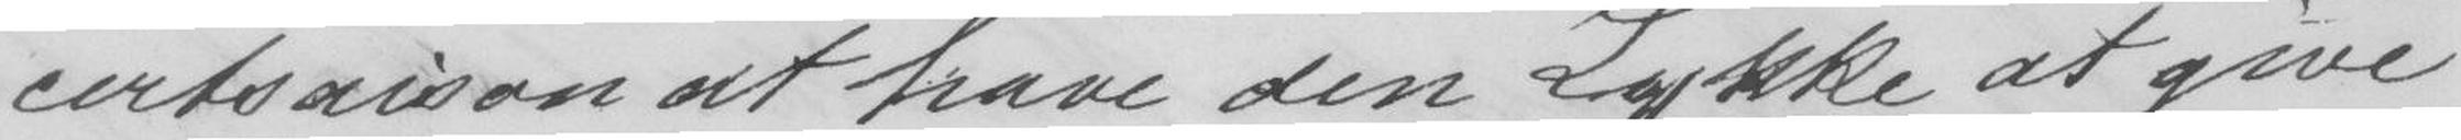certsaison at have den Lykke at give
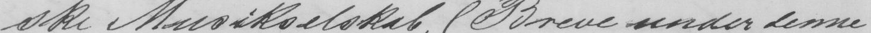ske Musikselskab, (Breve under denne
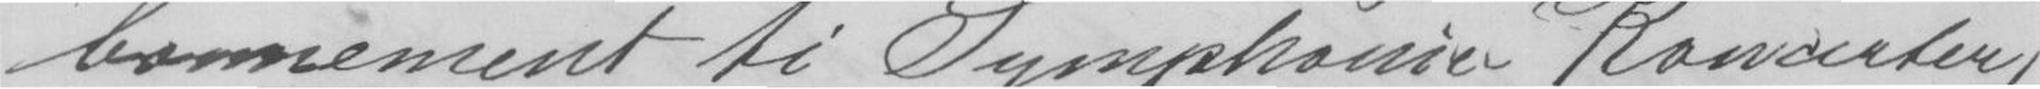bonnement to Symphonie Koncerter,
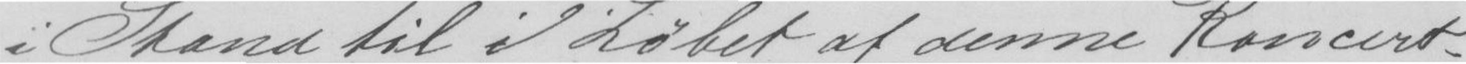i Stand til i Løbet af denne Koncert-
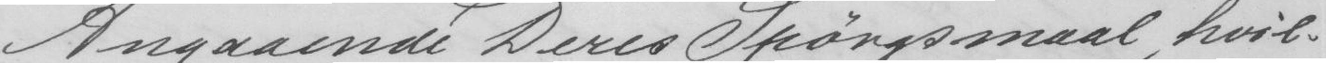Angaaende Deres Spørgsmaal, Hvil-
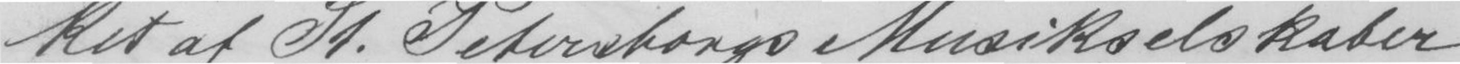ket af St.Petersborgs Musikselskaber
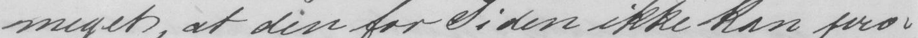meget, at den for Tiden ikke kan pro-
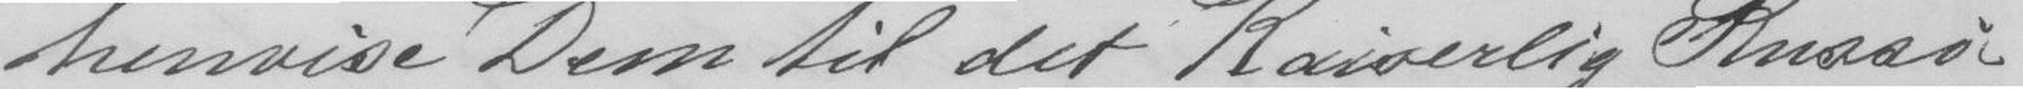henvise Dem til det Kaiserlig Russi-
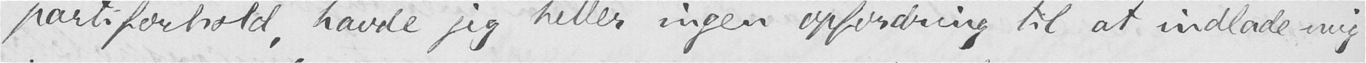partiforhold, havde jeg heller ingen opfordring til at indlade mig
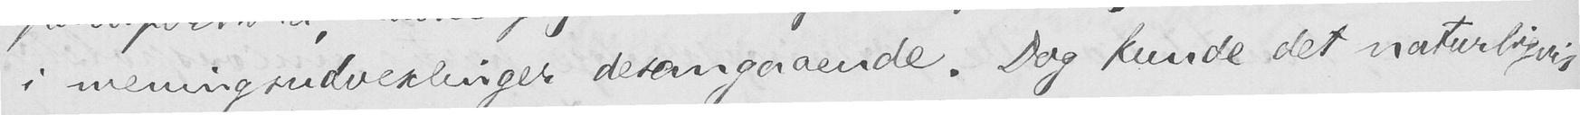i meningsudvexlinger desangaaende. Dog kunde det naturligvis
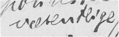væsentlige
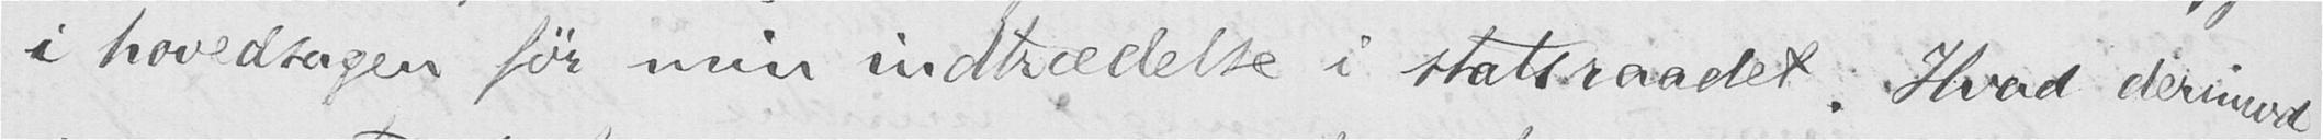i hovedsagen før min indtrædelse i statsraadet. Hvad derimod
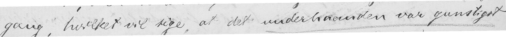gang, hvilket vil sige, at det underhaanden var gunstigst
